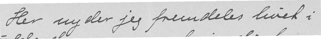Her nyder jeg fremdeles livet i
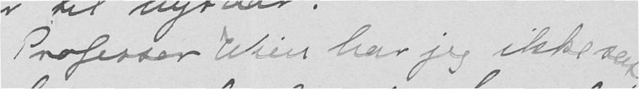Professor Wein har jeg ikke set
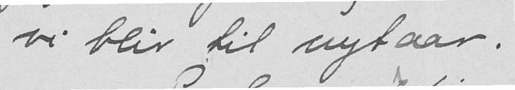vi blir til nytaar.
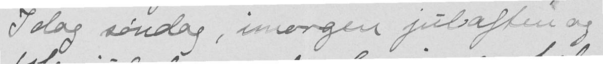Idag søndag, imorgen juliaften og
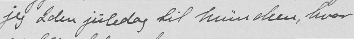jeg 2den juledag til Uninchen, hvor
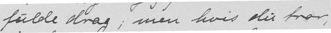fulde drag; men hvis du tror.
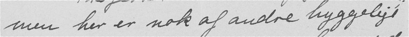men her er nok af andre hyggelige.
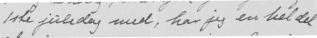1ste juledag med, har jeg en hel del
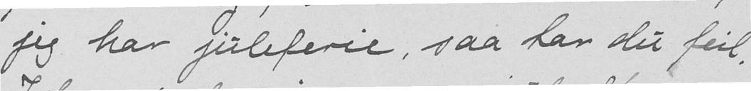jeg har juleferie, saa tar du feil.
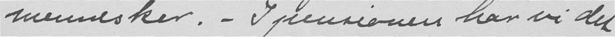mennsker. - I pensionen har vi det
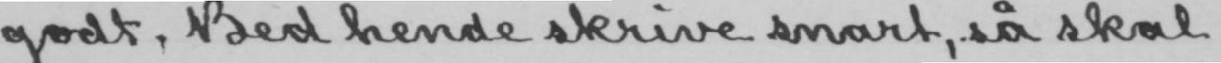godt. Bed hende skrive snart, så skal
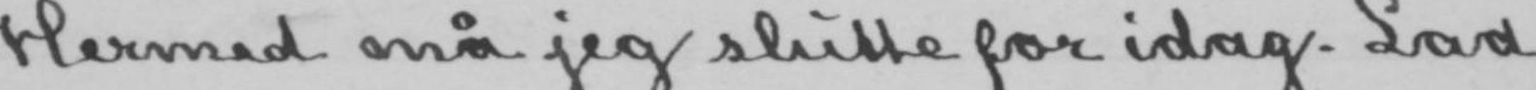Hermed må jeg slutte for idag. Lad
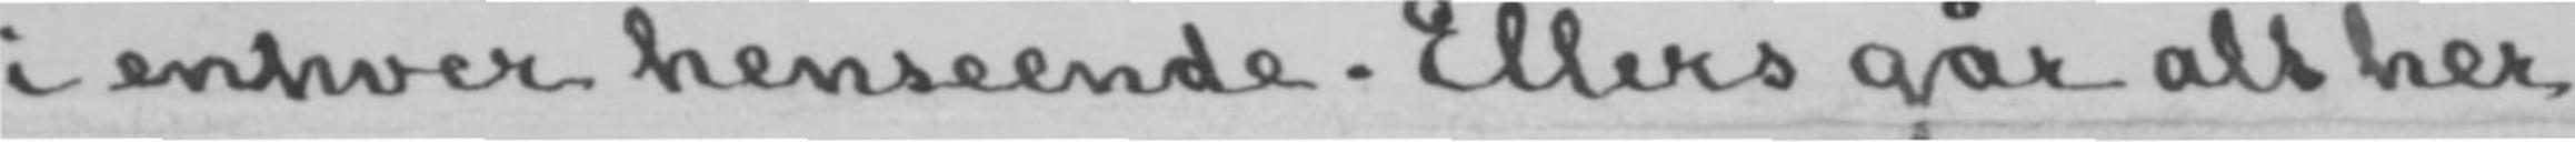i enhver henseende. Ellers går alt her
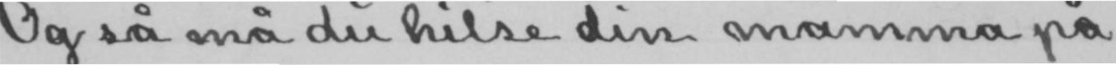Og så må du hilse din mamma på
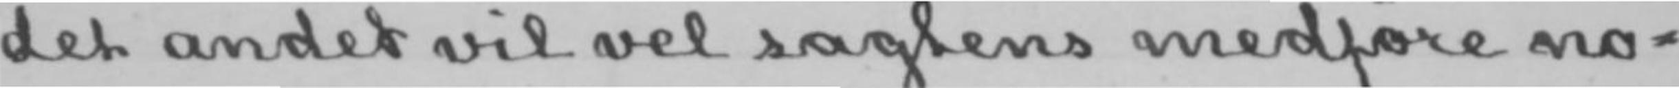det andet vil vel sagtens medføre no-
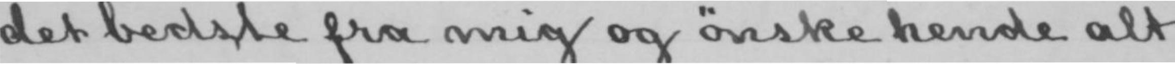det bedste fra mig og ønske hende alt
